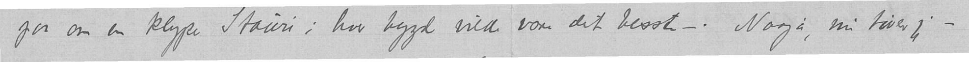paa om en klype Stauri i hver bygd vilde være det besste –. Naaja, nu tøver jeg -
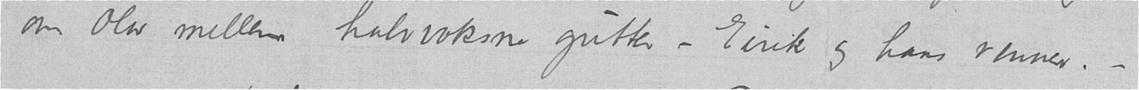om Olav mellem halvvoksne gutter – Eirik og hans venner. –
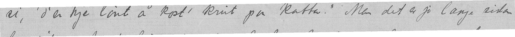si, "D'er 'kje lønt å kost' krut paa katta." Men det er jo længe siden
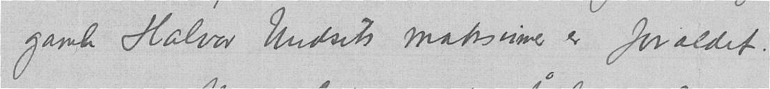gamle Halvor Undsets maksimer er forældet.
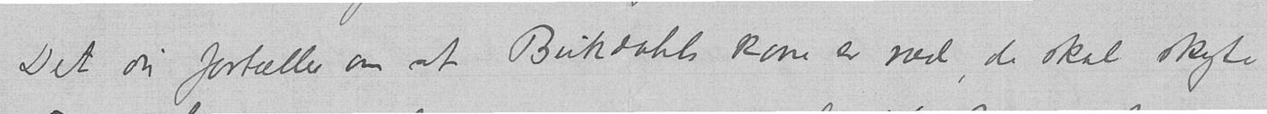Det du fortæller om at Bukdahls kone er ræd, de skal skyte
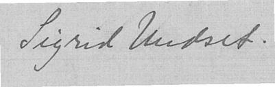Sigrid Undset.
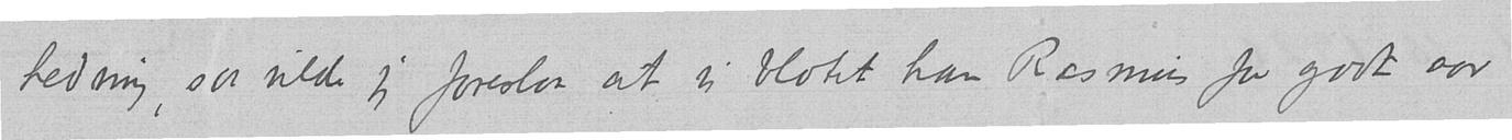hedning, saa vilde jeg foreslaa at vi blotet han Rasmus for godt aar
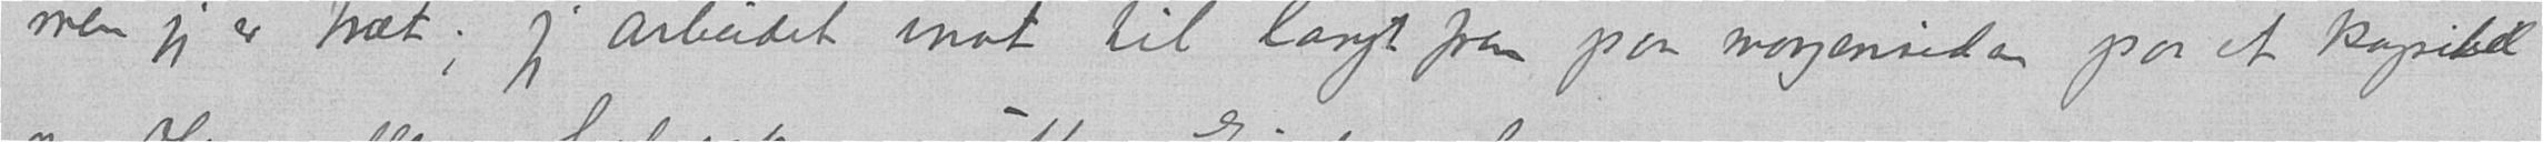men jeg er træt; jeg arbeidet inat til langt frem paa morgensiden paa et kapitel
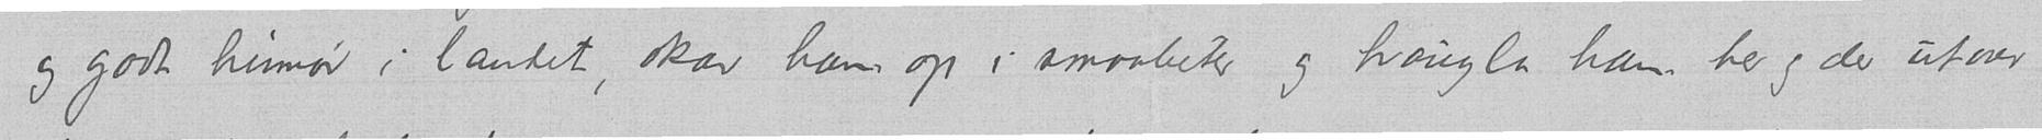og godt humør i landet, skar ham op i smaabeter og haugla ham her og der utover
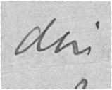din
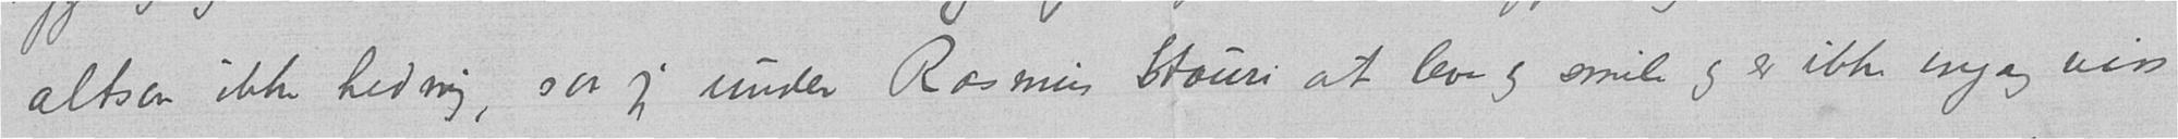altsaa ikke hedning, saa jeg under Rasmus Stauri at leve og smile og er ikke engang viss
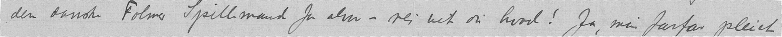den danske Folmer Spillemand for alvor – nei vet du hvad! Ja, min farfar pleiet
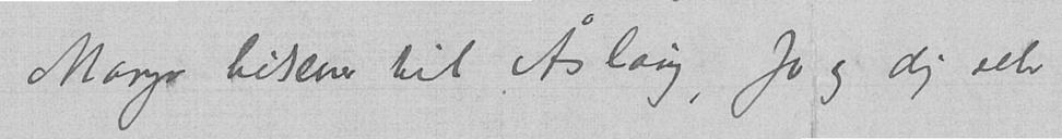Mange hilsener til Åslaug, Jo og dig selv
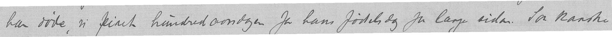han døde, vi feiret hundredaarsdagen for hans fødselsdag for længe siden. Saa kanske
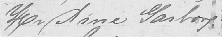Hr Arne Garborg
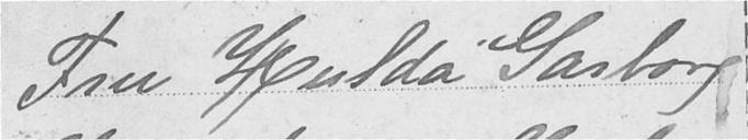Fru Hulda Garborg
